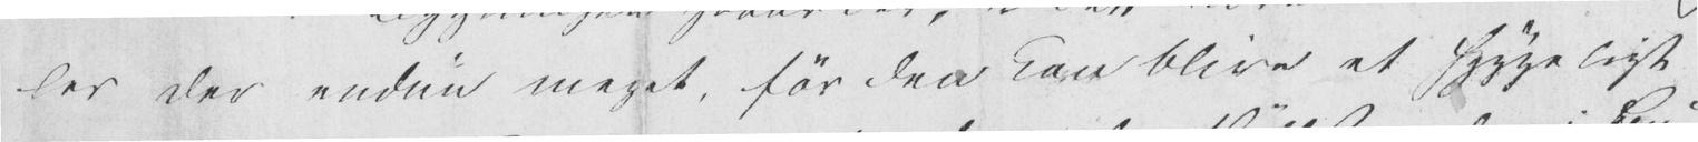ler der endnu meget, før den kan blive et hyggeligt
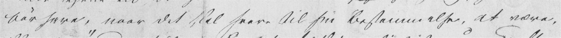bør have, naar det skal svare til sin Bestemmelse, at være,
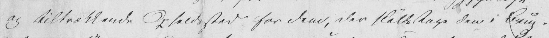og tiltrækkende Opholdssted for dem, der skulle tage den i Brug.
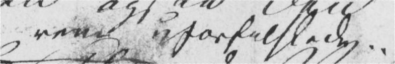rene uforfalskede
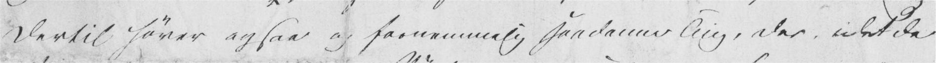Dertil hører ogsaa og fornemmelig saadanne Ting, der, idet de
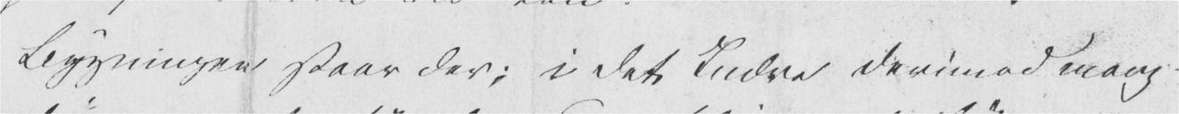Bygningen staar der; i det Indre derimod mang-
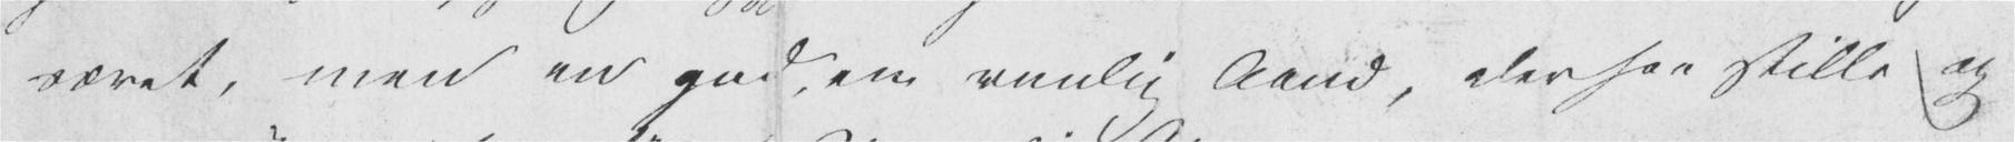været, men en god, en venlig Aand, der saa stille og
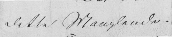dette Manglende.
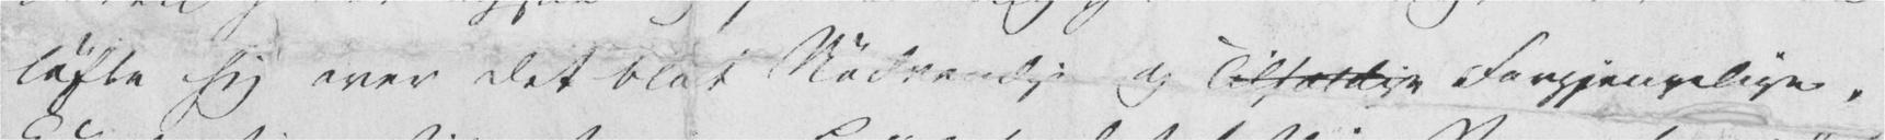løfte sig over det blot Nødvendige og Tilfældige Forgjengelige,
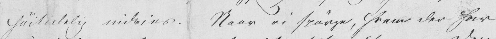høitidelig indvies. Naar vi spørge, hvem der har
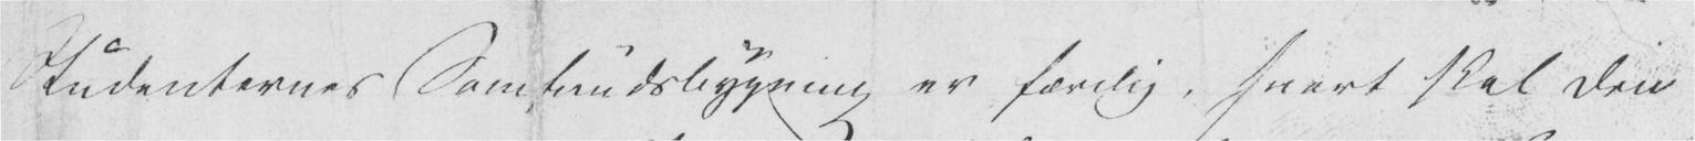Studenternes Samfundsbygning er færdig, snart skal den
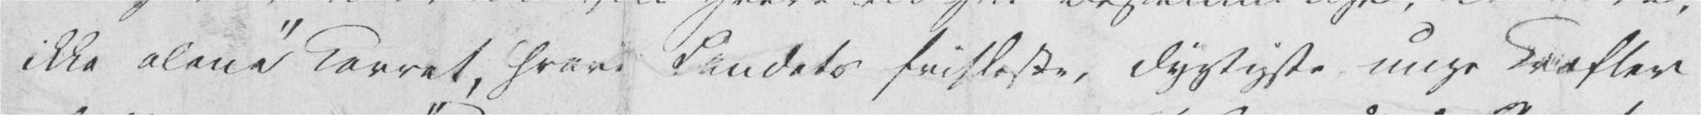ikke alene «Karret, hvor Landets friskeste, dygtigste unge Kræfter
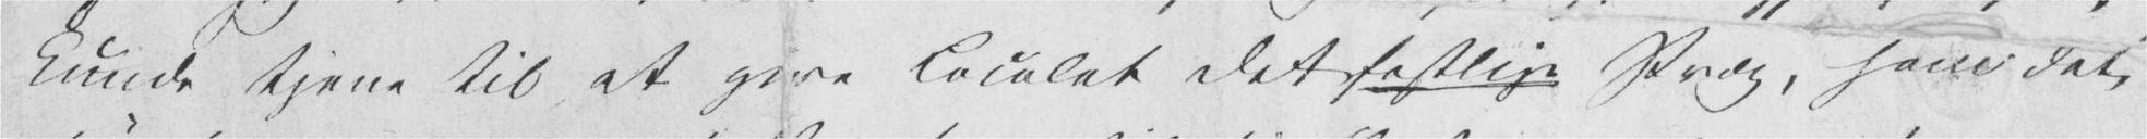kunde tjene til at give Localet det festlige Præg, som det
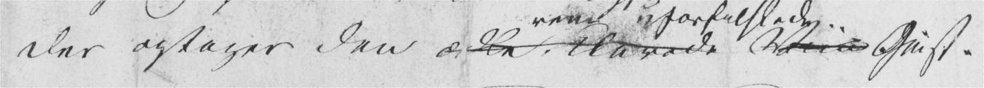der optager den ædle, klarede Viin Geist.
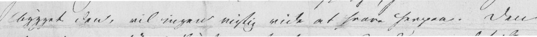bygget den, vil ingen rigtig vide at svare herpaa. Den
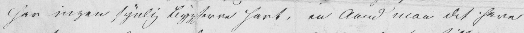har ingen synlig Bygherre havt, en Aand maa det have
